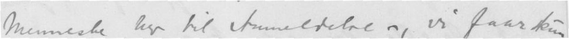Menneske her til Anmeldelse; vi faar kun
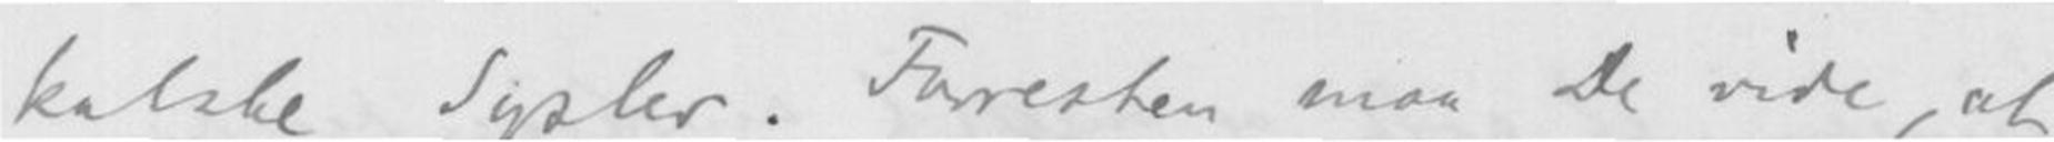kalske Sysler. Forresten maa Du vide, at
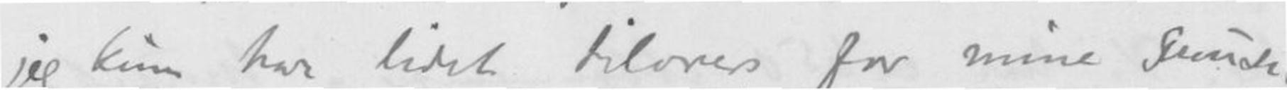jeg kun har lidt tilovers for mine Musi-
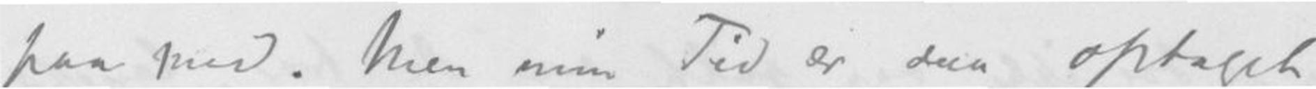paa med. Men min Tid er saa optaget
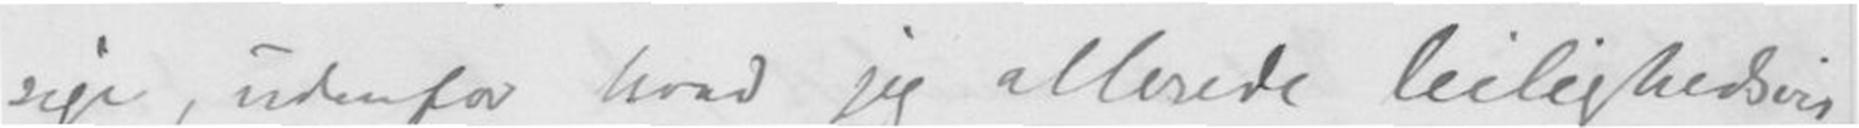sige, udenfor hvad jeg allerede leilighedsvis
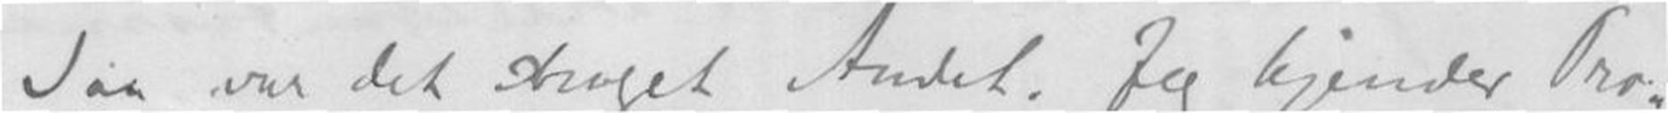Saa var det noget Andet. Jeg kjender Pro-
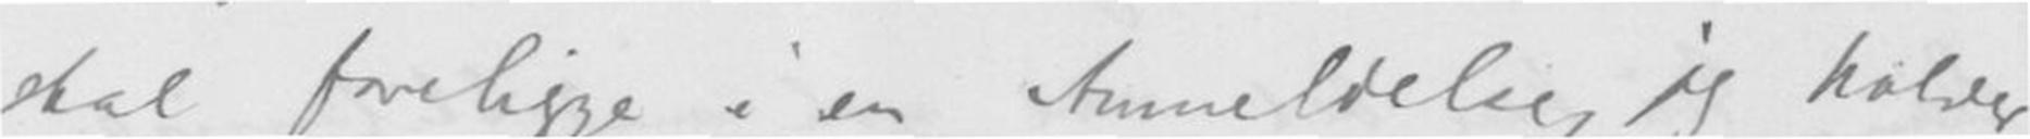skal foreligge i en Anmeldelse, jeg holder
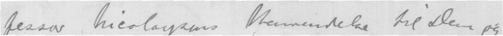fessor Nicolaysens Henvendelse til Dem og
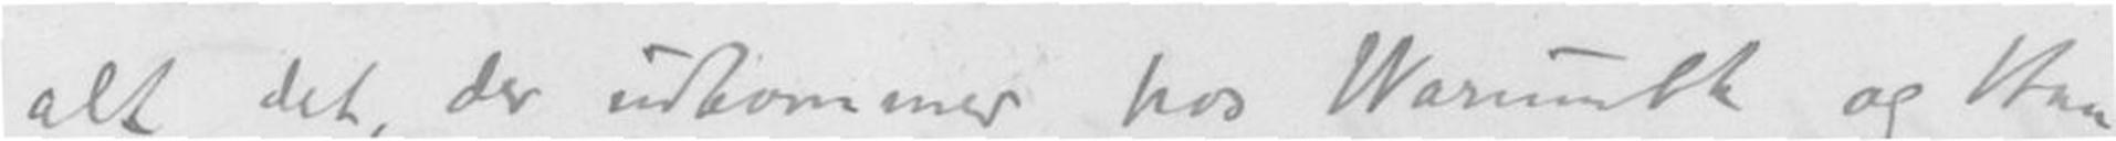alt det, de indkommer hos Warmuth og Haa-
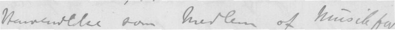Henvendelse som Medlem af Musikfor
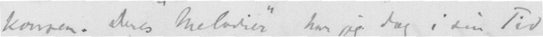konsen. Deres "Melodier" har jeg dog i sin Tid
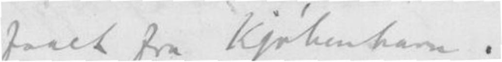faadt fra Kjøbenhavn.
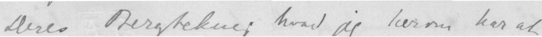Deres Bergtekne ; hvad jeg herom har at
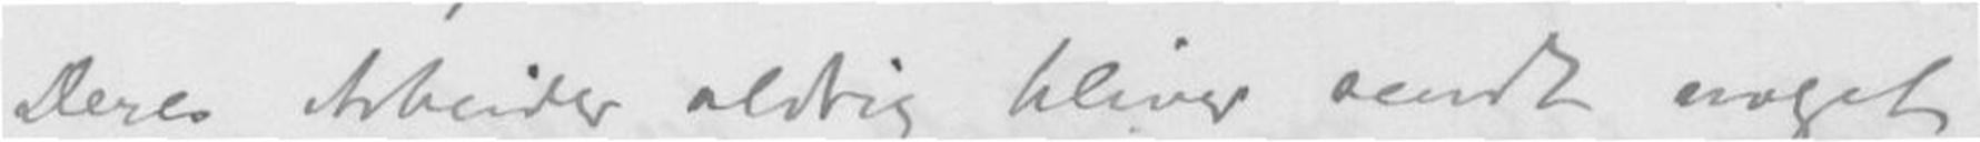Deres Arbeide aldtig blive sendt noget
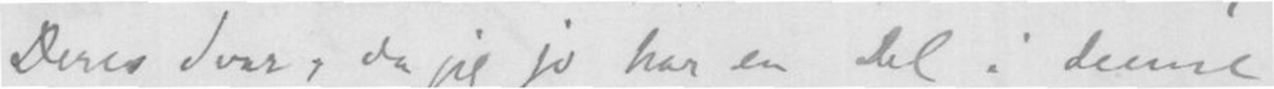Deres Svar, da jeg jo har en del i denne
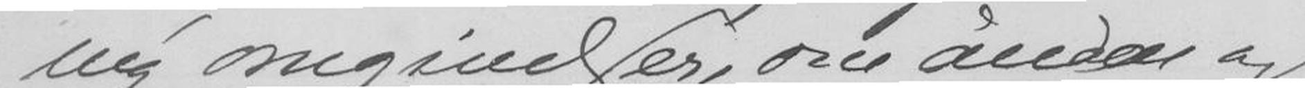ny omgivelser, om ånden og
